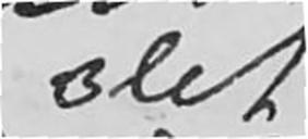slet
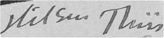Hilsen Thiis
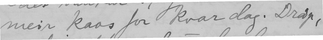meir kaos for kvar dag. Dråp,
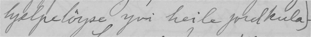hjelpelöyse yvi heile jordkula.
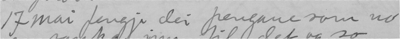17mai fengji dei pengane som no
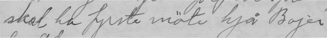skal ha fyrste möte hjå Bojer
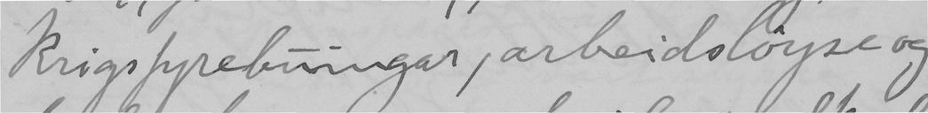krigstypebuingar, arbeidslöyse og
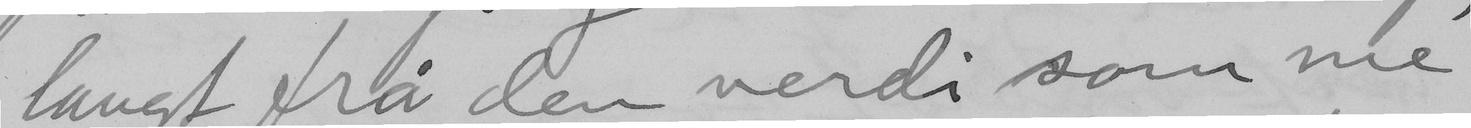langt frå den verdi som me
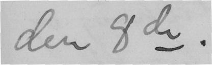den 8de.
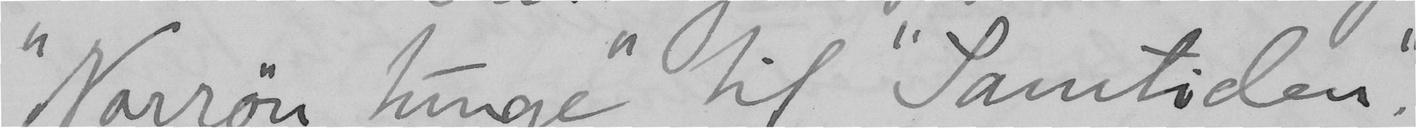"Nynorsk tunge" til "Samtiden".
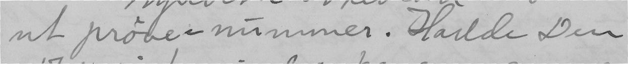ut pröve-nummer. Hadde den
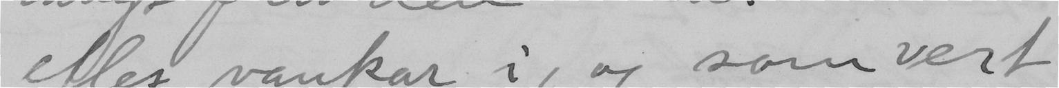elles vankar i, og som vert
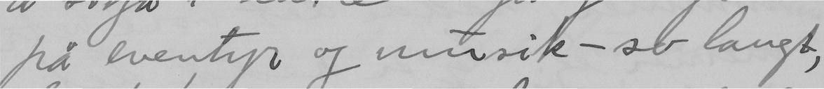på eventyr og musik – so langt,
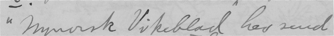"Nynorsk Vikeblad" hev send
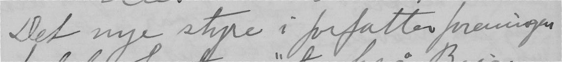Det nye styre i forfatterforeningen
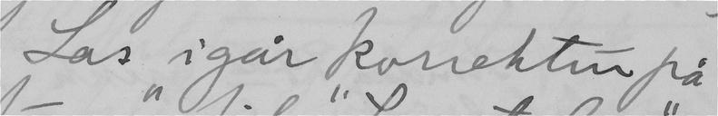Las igår korrektur på
